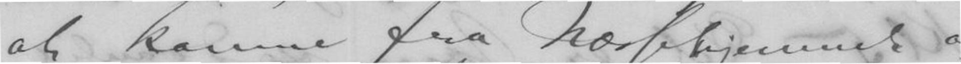at komme fra Næssethjemmet og
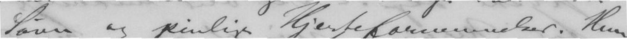Søvn og pinlige Hjertefornemmelser. Hun
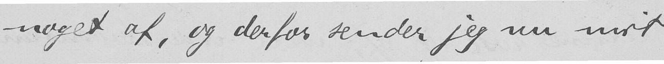noget af, og derfor sender jeg nu mit
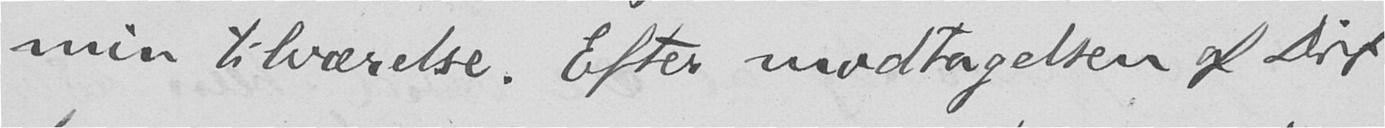min tilværelse. Efter modtagelsen af Dit
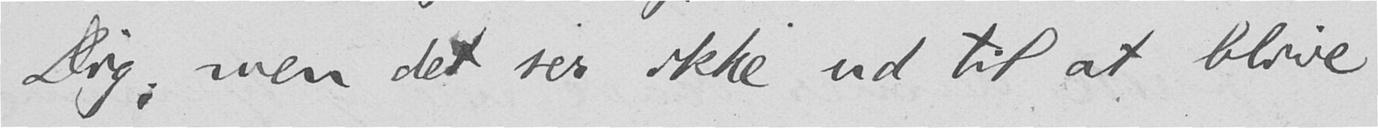Dig; men det ser ikke ud til at blive
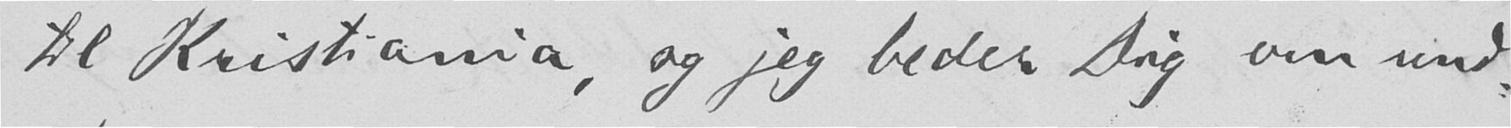til Kristiania, og jeg beder Dig om und-
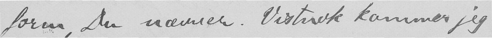form, Du nævner. Vistnok kommer jeg
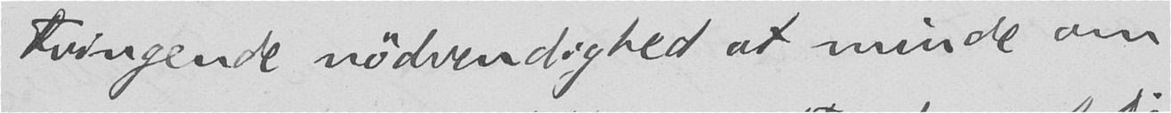tvingende nødvendighed at minde om
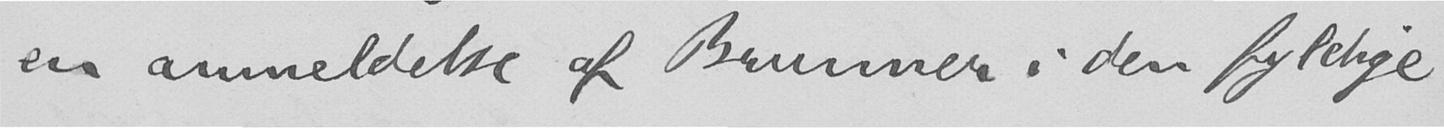en anmeldelse af Brunner i den fyldige
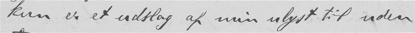kun er et udslag af min ulyst til uden
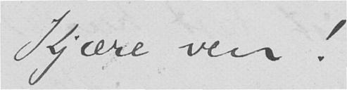Kjære ven!
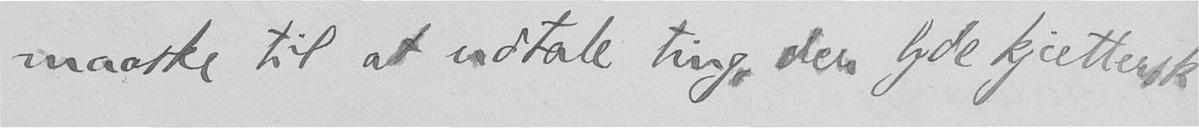maaske til at udtale ting, der lyde kjættersk
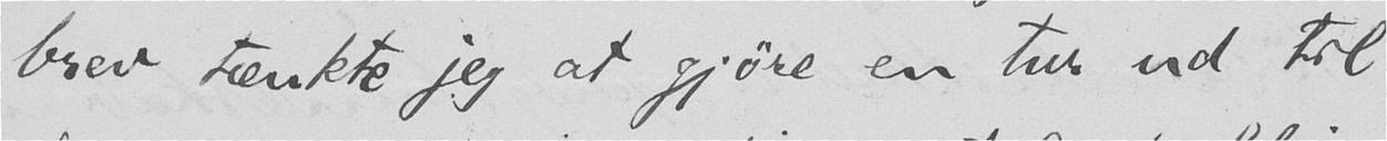brev tænkte jeg at gjøre en tur ud til
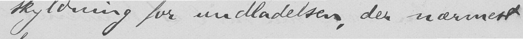skyldning for undladelsen, der nærmest
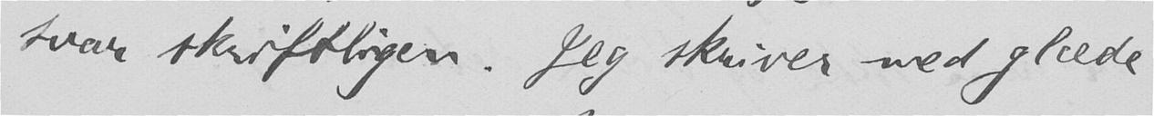svar skriftligen. Jeg skriver med glæde
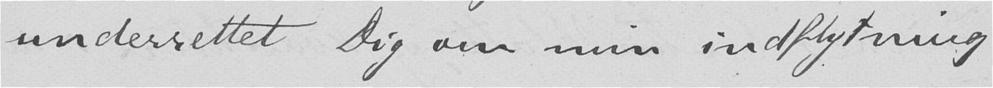underrettet Dig om min indflytning
